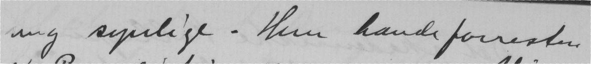ung synlige. Hun havde forresten
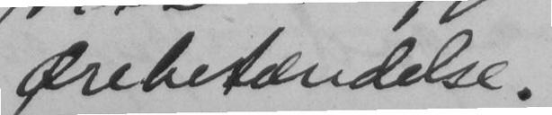Ørebetændelse.
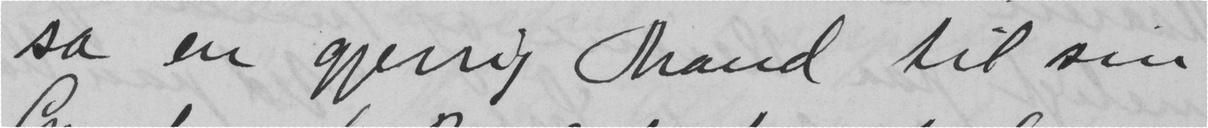sa en gjerrig Mand til sin
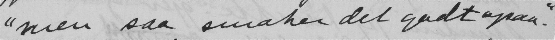"men saa smaker det godt ogsaa".
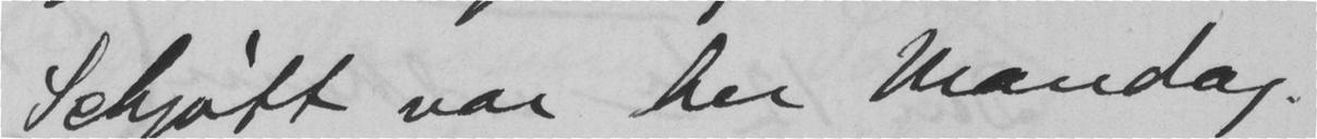Schjøtt var her Mandag.
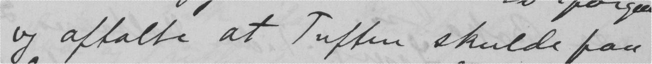og aftalte at Tuften skulde faa
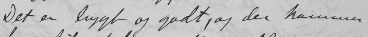Det er trygt og godt, og der kommer
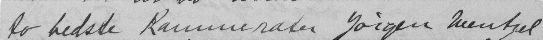to bedste Kammerater Jørgen Wentzel
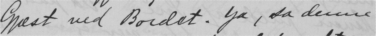Gjæst ved Bordet. Ja, sa denne
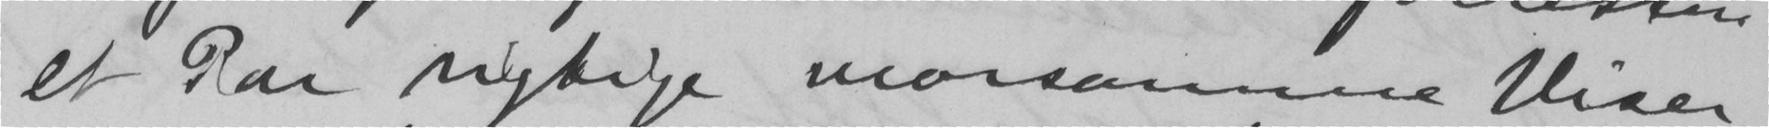et Par rigtige morsomme Viser
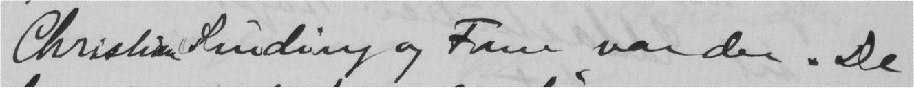Christian Sinding og Frue var der. De
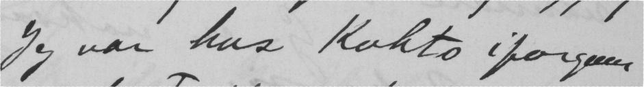Jeg var hos Kohts iforgaar
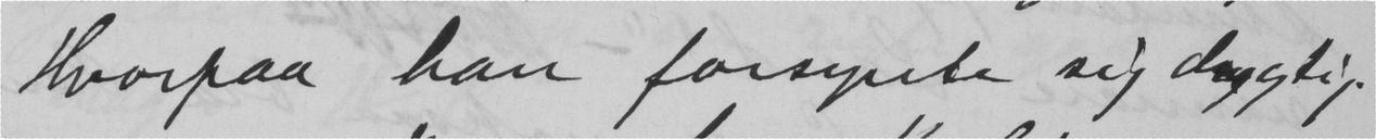Hvorpaa han forsynte sig dygtig.
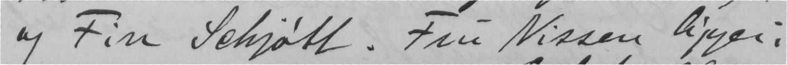og Fin Schjøtt. Fru Nissen ligger i
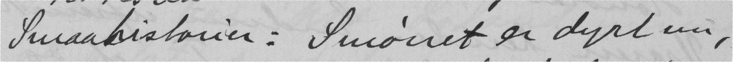Smaahistorier: Smøret er dyrt nu,
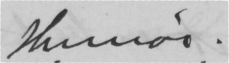Humør.
